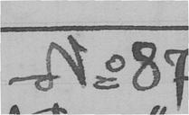No 87
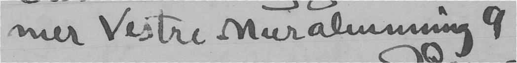mer Vestre Muralmming 9
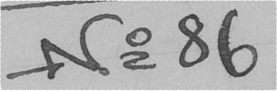No 86
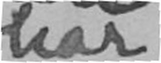har
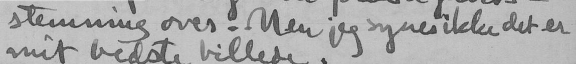stemning over. Men jeg synes ikke det er
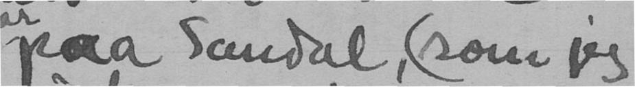paa Sandal, (som jeg
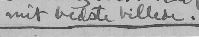mit bedste billede.
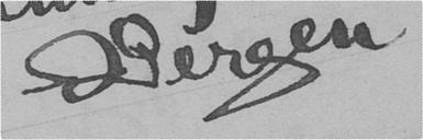Bergen
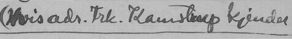(Hvis adr. Frk Kamstrup kjender
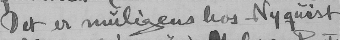Det er muligens hos Nyquist
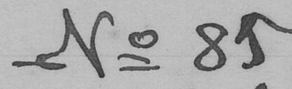No 85
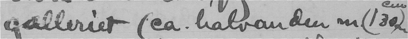galleriet (ca. halvanden m (130)
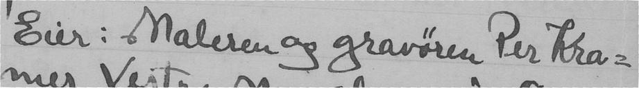Eier: Maleren og gravøren Per Kra-
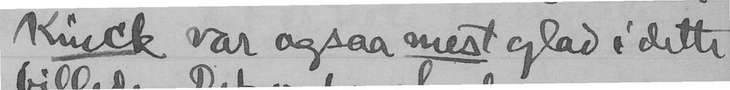Kinck var ogsaa mest glad i dette
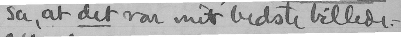sa, at det var mit bedste billede. -
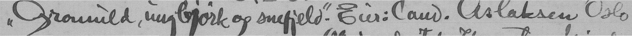"Gromuld, ung bjørk op snefjeld". Eier Cand. Aslaksen Oslo
